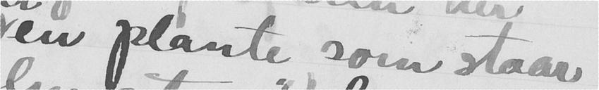en plante som staar
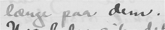længe paa dem.
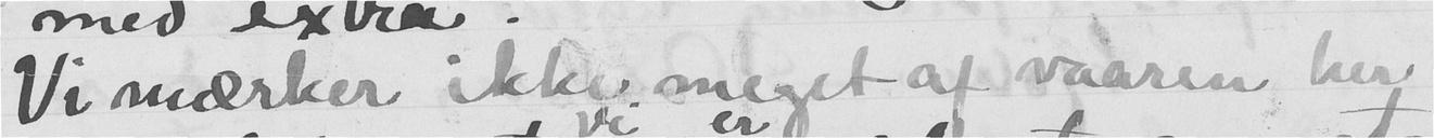Vi mærker ikke meget af vaaren her
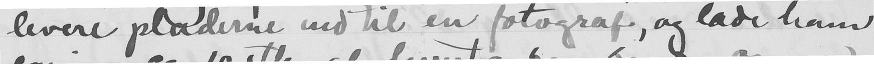levere pladerne ind til en fotograf, og lade ham
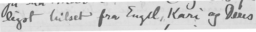ligst hilset fra Engel, Kari og Deres
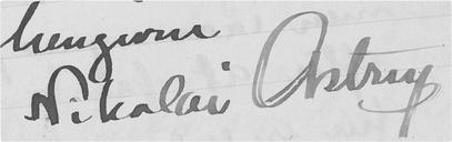Nikolai Astrup
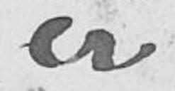er
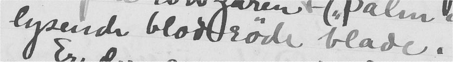lysende blodrøde blade.
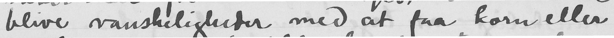blive vanskeligheder med at faa korn eller
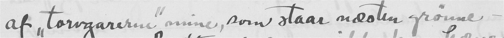af "torvgarerne" mine, som staar næsten grønne -
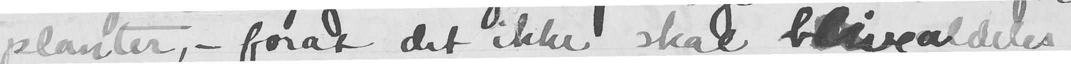planter, - forat det ikke skal blive aldeles
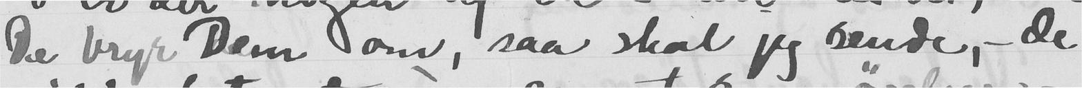De bryr Dem som, saa skal jeg sende, - de
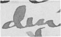den
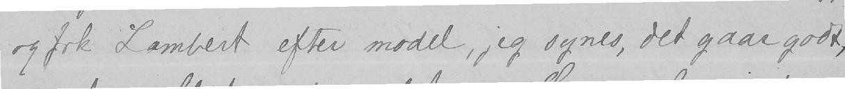og frk Lambert efter model, jeg synes, det gaar godt,
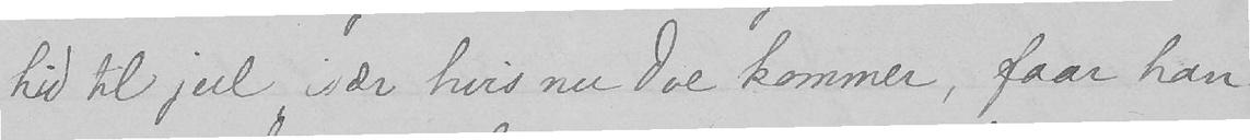hid til jul især hvis Ove kommer, faar han
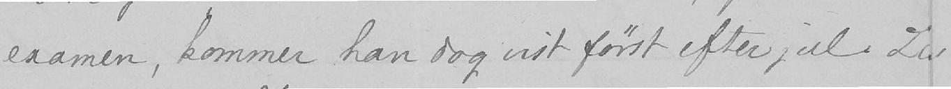examen, kommer han dog vist først efter jul. Lev
